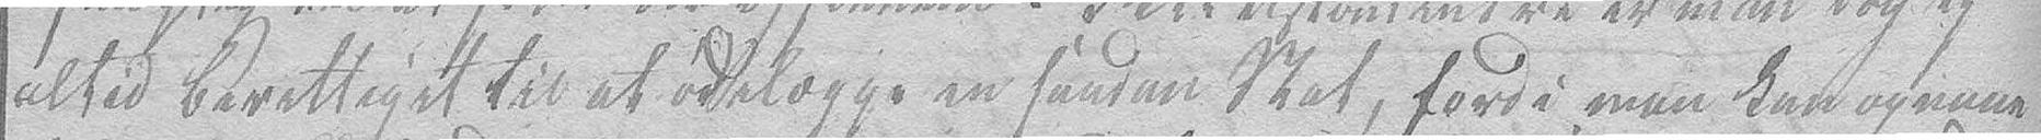altid berettiget til at ødelægge en saadan Stat, fordi man kan opnaae
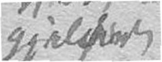gjelder
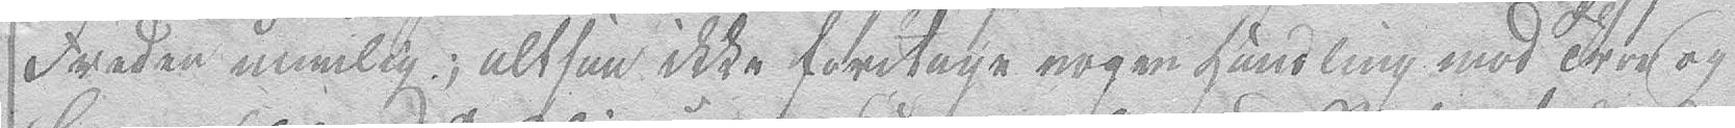Freden umulig; altsaa ikke foretage nogen Handling mod Troe og
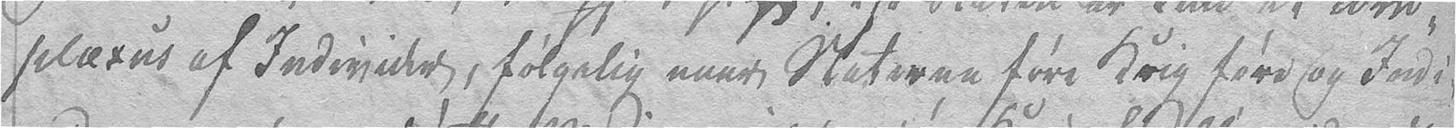plexus af Individer, følgelig naar Staterne føre Krig føre og Indi-
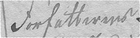Fortatterens
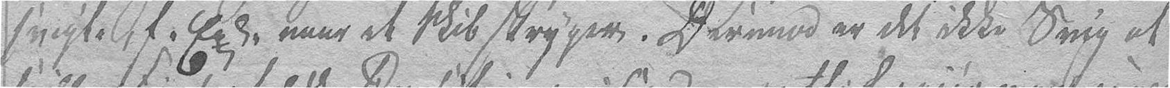svigte, f. Exl. naar et Skib stryger. Derimod er det ikke Svig at
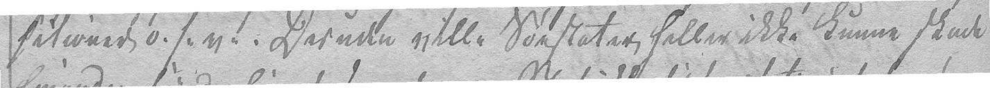sitioner o:s:v:. Desuden ville Søestater heller ikke kunne skade
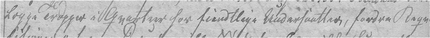lægge Tropper i Qvarteer hos fiendtlige Undersaatter, fordre Reqvi-
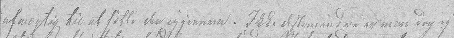afmægtig til at sætte den igjennem. Ikke destomindre er man dog ey
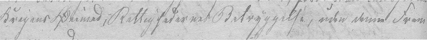Krigens Øiemed; Rettighedernes Betryggelse, uden denne Frem-
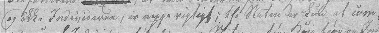og ikke Individerne, er neppe rigtigt; thi Staten er kun et com-
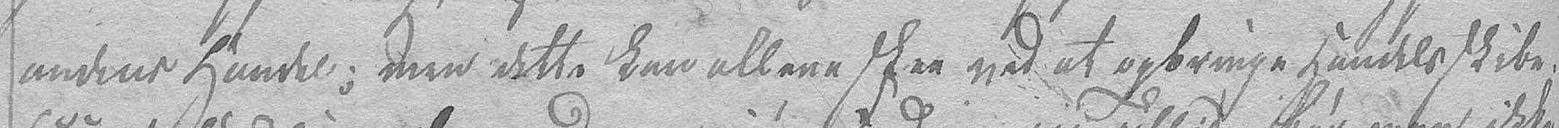andens Handel; men dette kan allene skee ved at opbringe Handelsskibe.
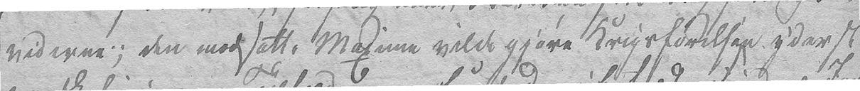viderne; den modsatte Maxime vilde gjøre Krigsførelsen yderst
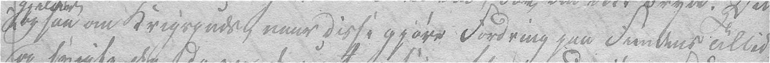ogsaa om Krigsgods, naar disse gjøre Fordring paa Fiendens Tillid
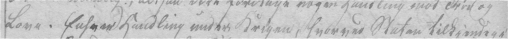Love. Enhver Handling under Krigen, hvorved Staten tilkjendegi-
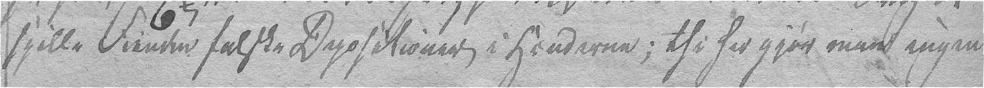spille Fienden falske Depositioner i Hænderne; thi her gjør man ingen
